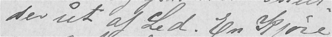der ut af Led. En Kjøre-
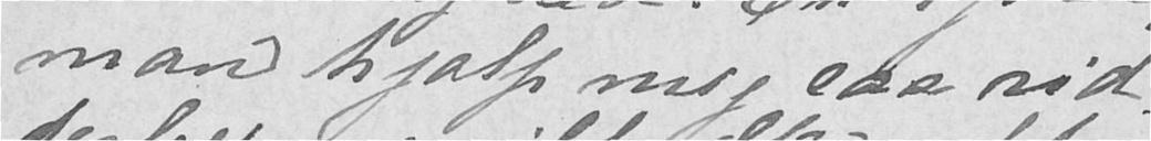mand hjalp mig saa rid-
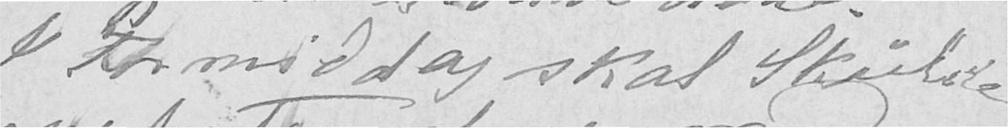I Formiddag skal Skulde-
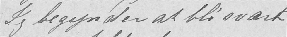Jeg begynder at bli svært
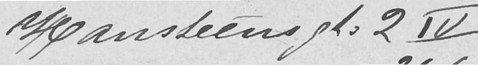Hansteensgt. 2 IV
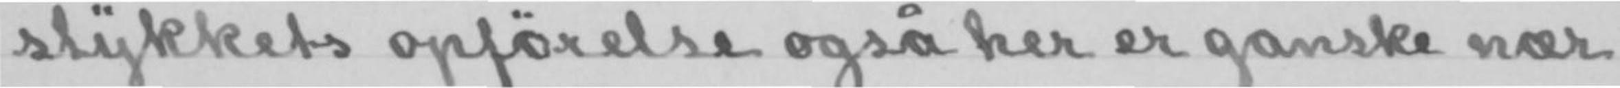stykkets opførelse også her er ganske nær
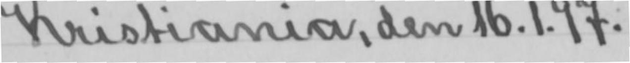Kristiania, den 16.1.97.
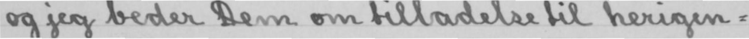og jeg beder Dem om tilladelse til herigen-
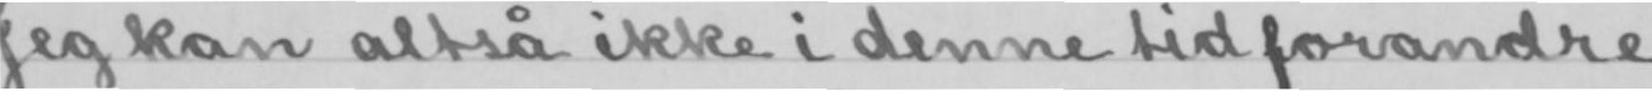Jeg kan altså ikke i denne tid forandre
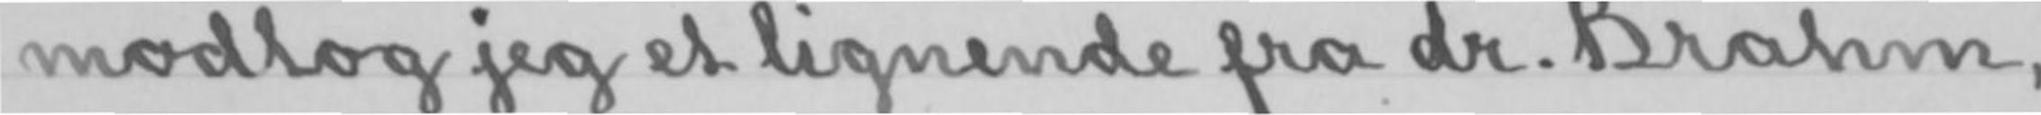modtog jeg et lignende fra dr. Brahm,
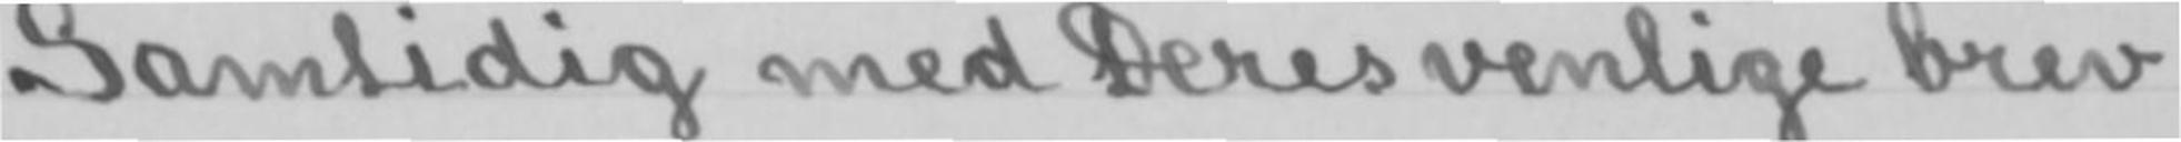Samtidig med Deres venlige brev
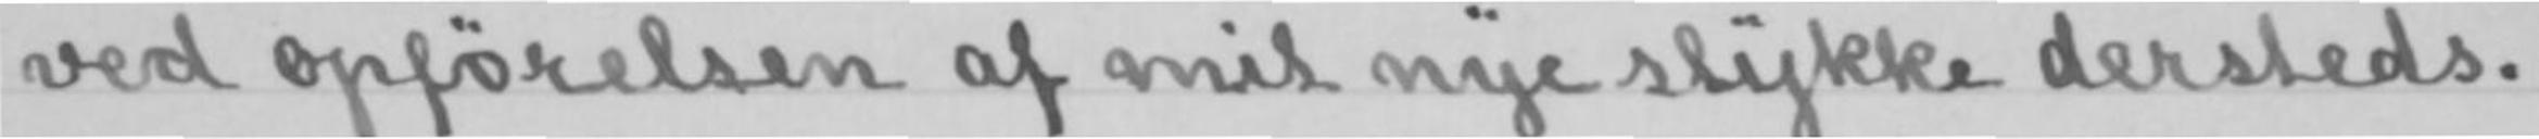ved opførelsen af mit nye stykke dersteds.
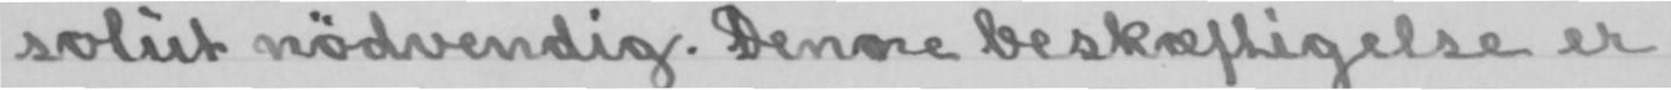solut nødvendig. Denne beskæftigelse er
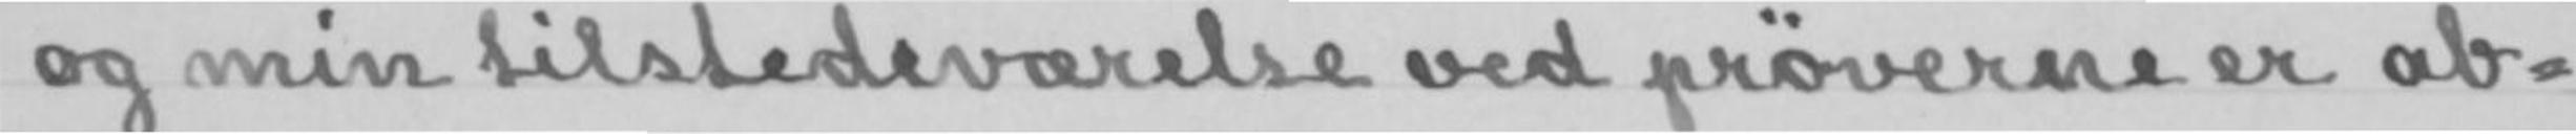og min tilstedeværelse ved prøverne er ab-
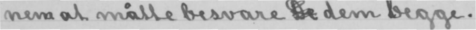nenm at måtte besvare De dem begge.
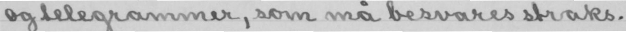og telegrammer, som må besvares straks.
